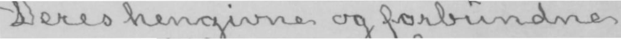Deres hengivne og forbundne
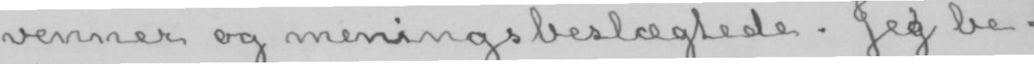venner og meningsbeslægtede. Jeg be-
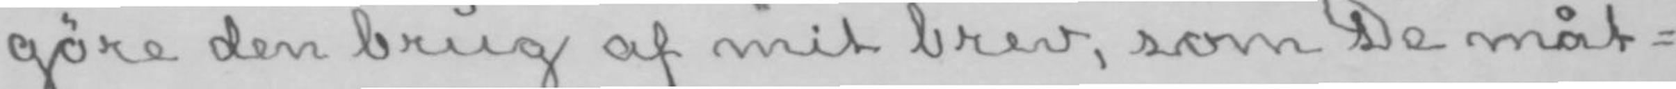gøre den brug af mit brev, som De måt-
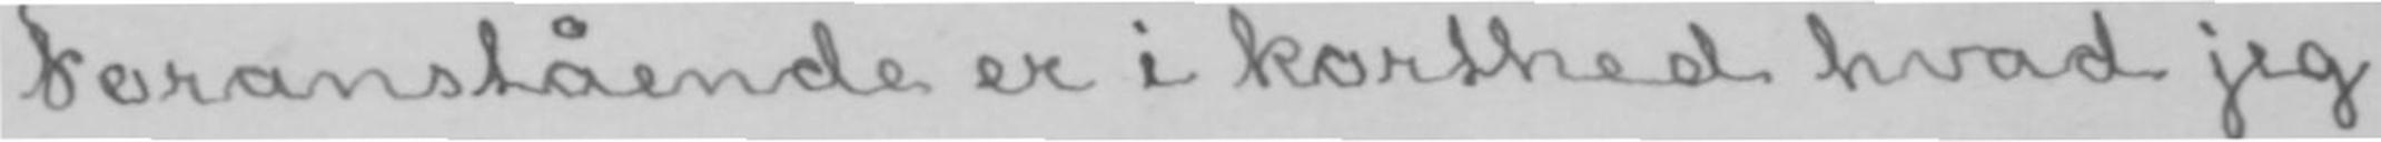Foranstående er i korthed hvad jeg
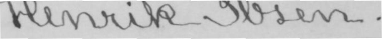Henrik Ibsen.
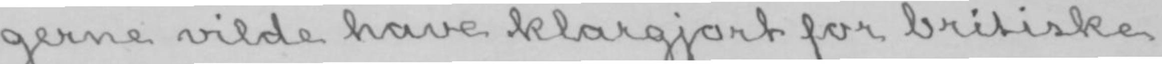gerne vilde have klargjort for britiske
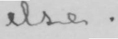else.
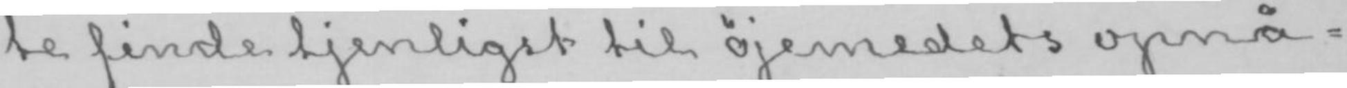te finde tjenligst til øjemedets opnå-
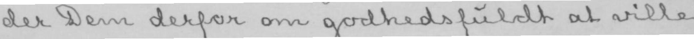der Dem derfor om godhedsfuldt at ville
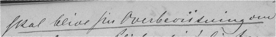skal blive sin Overbeviisning om
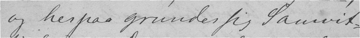og herpaa grunder sig Samvit-
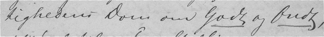tighedens Dom om Godt og Ondt,
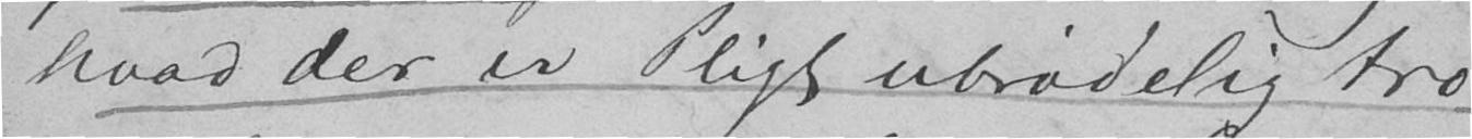hvad der er Pligt ubrødelig tro,
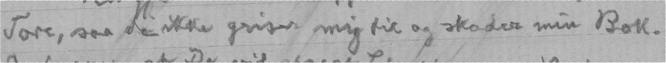Tore, saa de ikke griser mig til og skader min Bok.
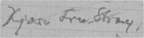Kjære Fru Stray,
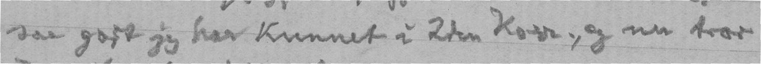saa godt jeg har kunnet i 2den Korr., og nu tror
